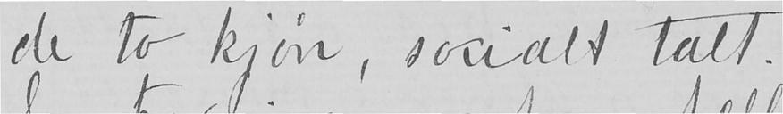de to kjøn, socialt talt.
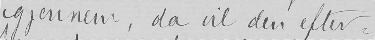igjennem, da vil den efter-
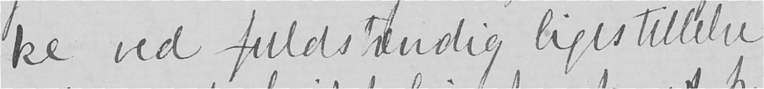ke ved fuldstændig ligestillelse
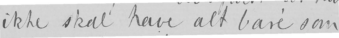ikke skal have alt bare som
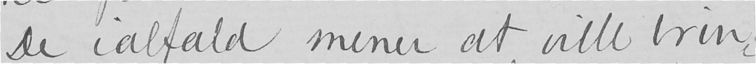De ialfald mener at ville brin-
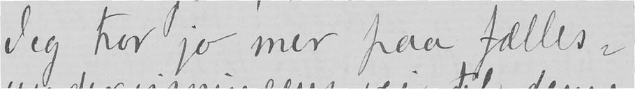Jeg tror jo mer paa fælles-
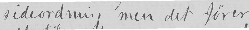sideordning, men det fører
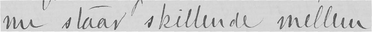nu staar skillende mellem
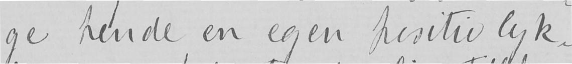ge hende en egen positiv lyk-
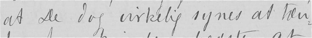at De dog virkelig synes at tæn-
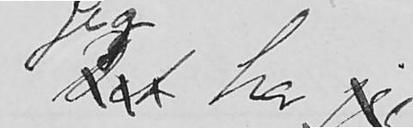Det har jeg
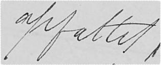opfattet
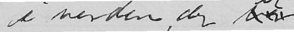i verden, der var
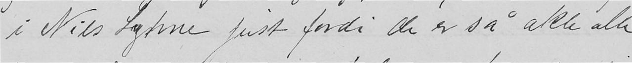i Nils Lyhne just fordi de er så ækle alle
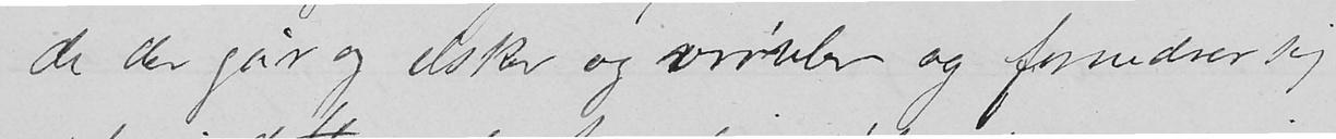de der går og elsker og vrøvler og fornedrer sig
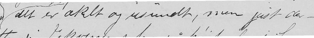det er ækelt og usundt, men just der-
